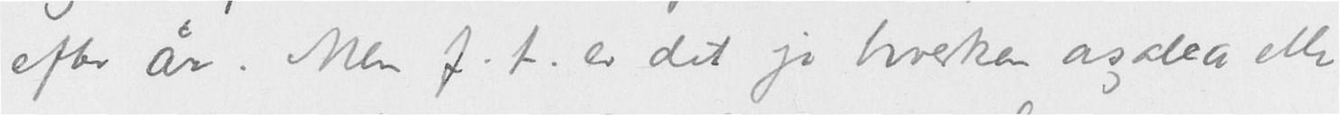efter år. Men f.t. er det jo hverken azalea eller
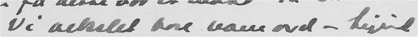Vi vekslet bare noen ord - Sigrid
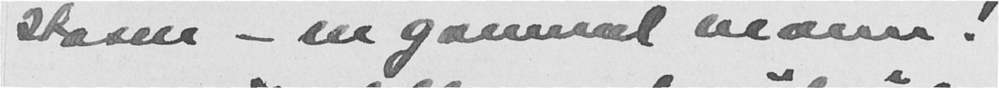stasen - en gammel mann!
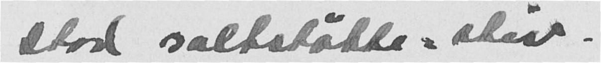stod saltstøtte-stiv.
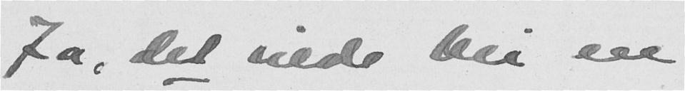Ja, det vilde bli en
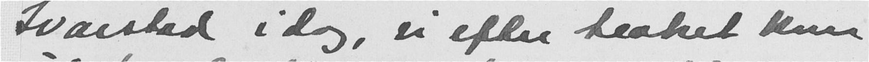Svarstad i dag, vi efter teatret kom
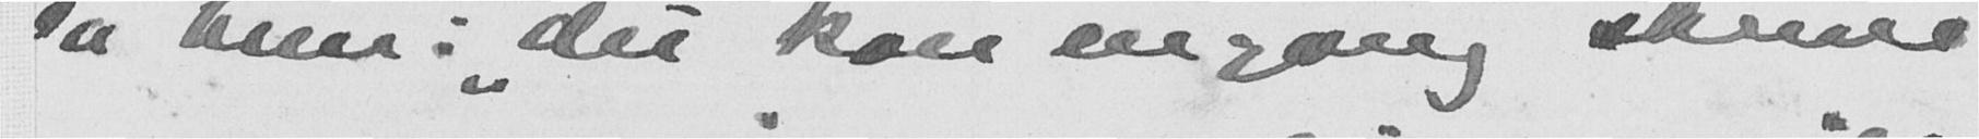sa hun: du kan engang skrive
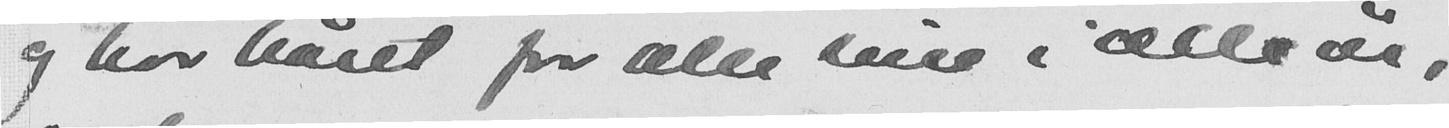og har båret for alle sine i alle år,
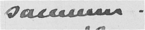sammen.
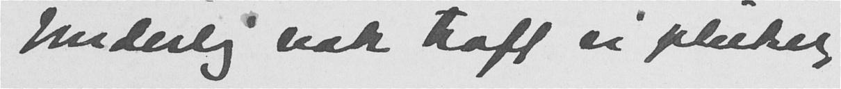Underlig nok traff vi plutselig
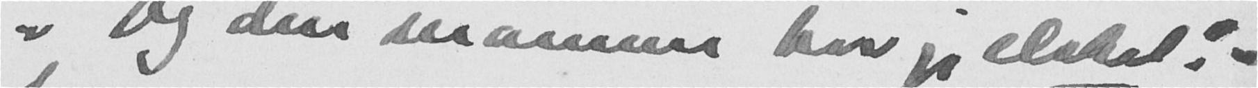"Og den mannen har jeg elsket:" -
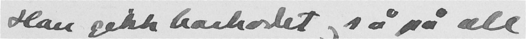Han gikk barhodet og så på all
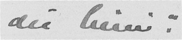du Nini."
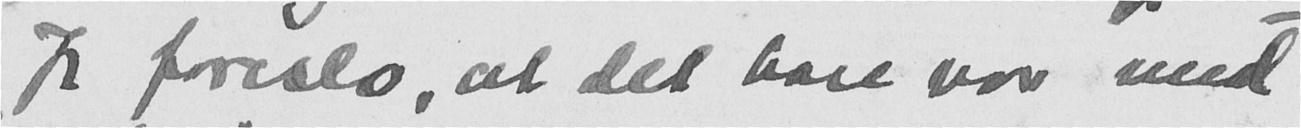Jeg foreslo, at det bare var med
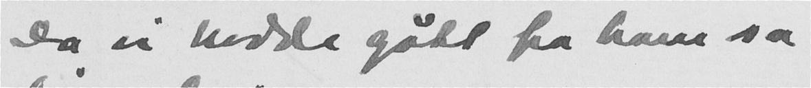Da vi hadde gått fra ham sa
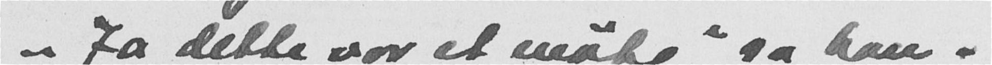"Ja dette var et møte" sa han.
